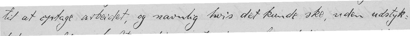til at optage arbeidet, og navnlig hvis det kunde ske, uden udstyk-
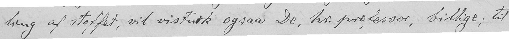ling af stoffet, vil vistnok ogsaa De, hr. professor, billige; til
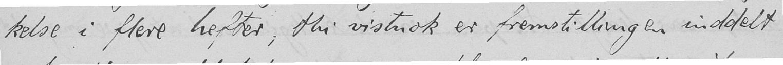kelse i flere hefter; thi vistnok er fremstillingen inddelt
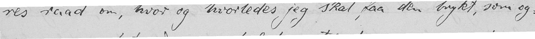res raad om, hvor og hvorledes jeg skal faa den trykt, som og-
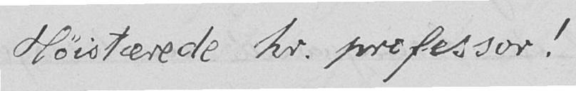Høistærede hr. professor!
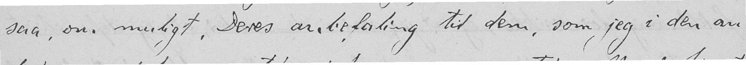saa, om muligt, Deres anbefaling til dem, som jeg i den an-
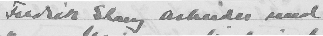Fredrik Stang arbeider med
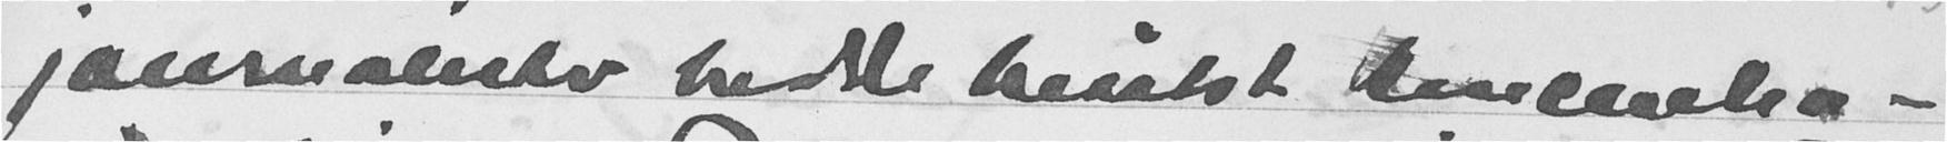journalister hadde besøkt kiccieha -
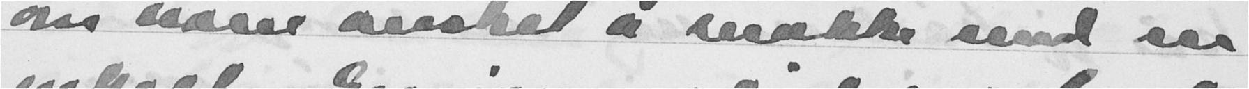om noen ønsket å snakke med en
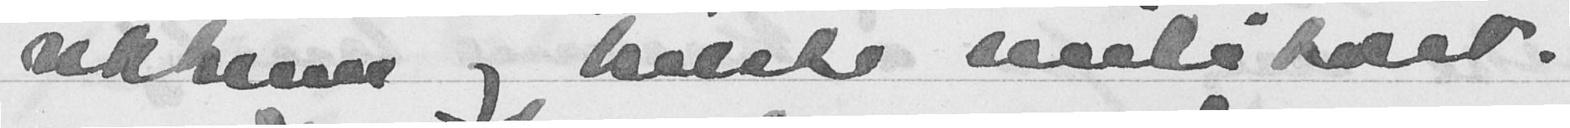rekkene og hilste militært.
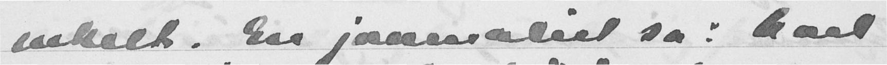enkelt. En journalist sa: Karl
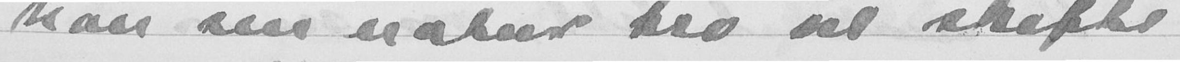han sin natur tro vil skifte
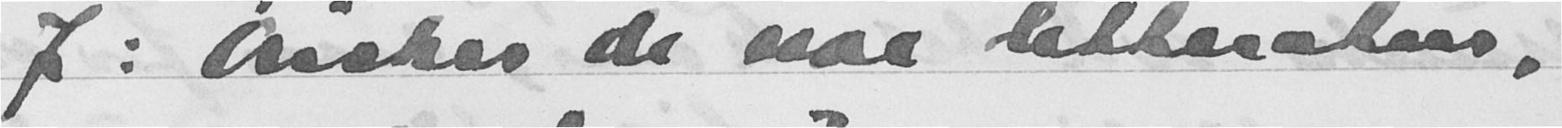J. Ønsker de noe litteratur,
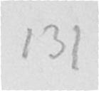131
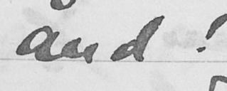ånd!
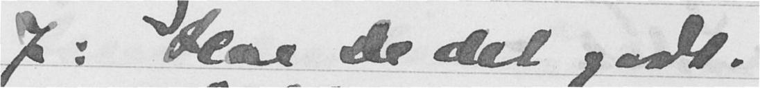J. Har De det godt.
